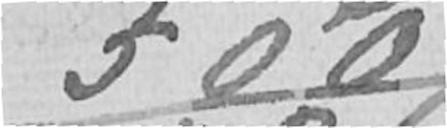500
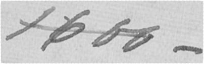1600-
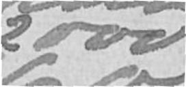2000
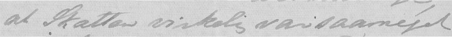at Skatten virkelig var saameget
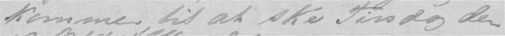kommer til at ske Tirsdag den
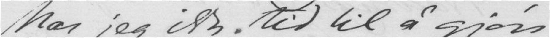har jeg ikke tid til å gjøre
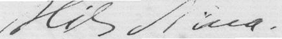Hils Nina.
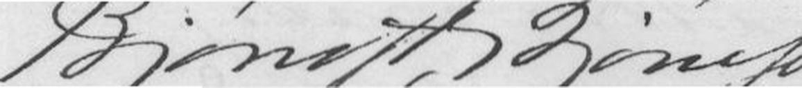Bjørnst. Bjørnson
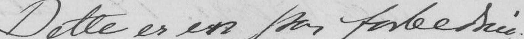Dette er en stor forbedring;
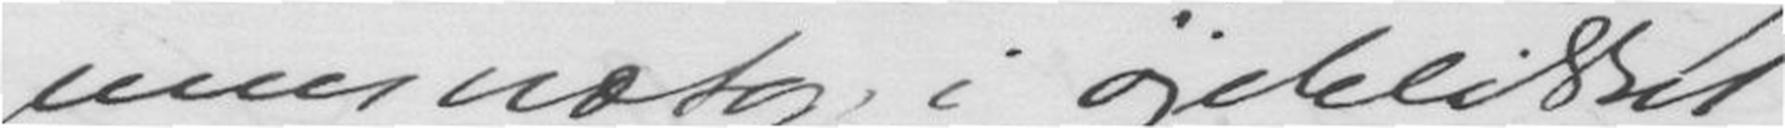men nætop i øjeblikket
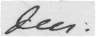den!
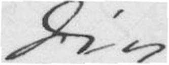Din
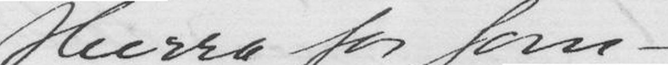Hurra for som-
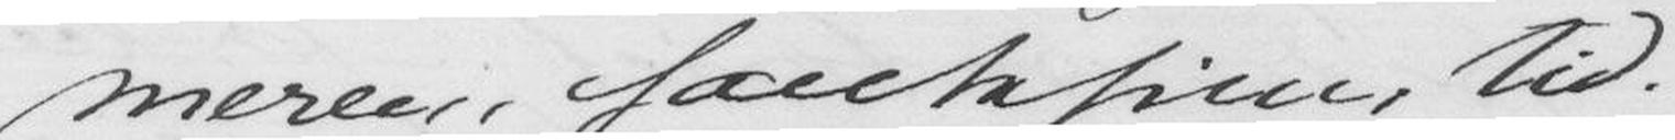meren fantasiens tid!
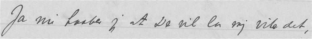Ja nu haaber jeg at De vil la mig vite det,
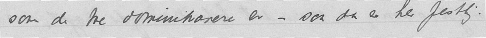som de tre dominikanere er – saa da er her festlig.
